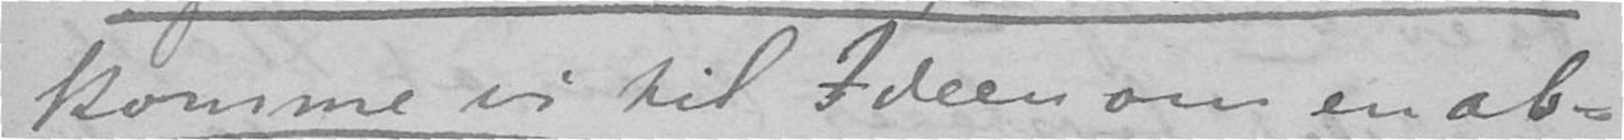komme vi til Ideen om en ab-
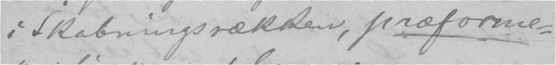i Skabningsrækken, præforme-
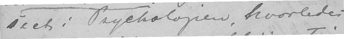seet i Psychologien, hvorledes
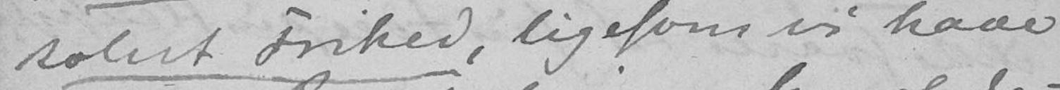solut Frihed, ligesom vi have
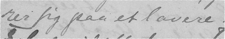rer sig paa et lavere.
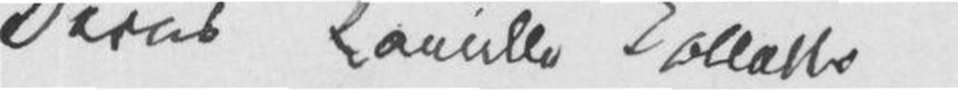Deres Camilla Collett.
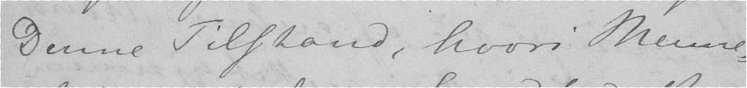Denne Tilstand, hvori Menne-
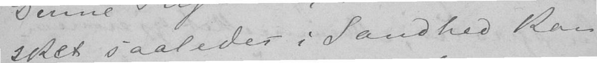sket saaledes i Sandhed kan
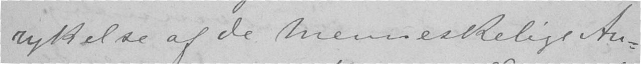rykkelse af de menneskelige An-
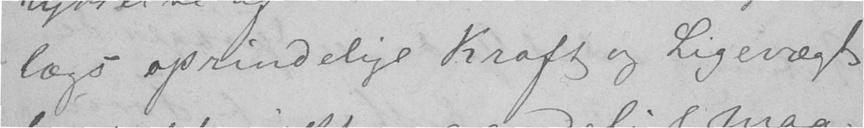lægs oprindelige Kraft og Ligevægt
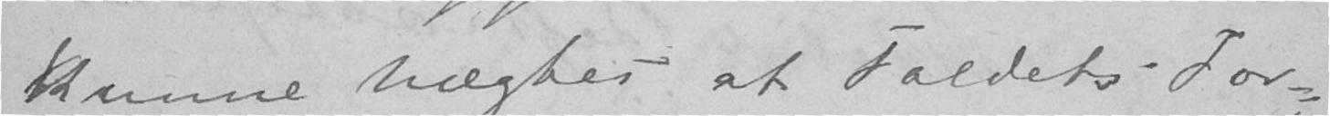kunne nægtes at Faldets For-
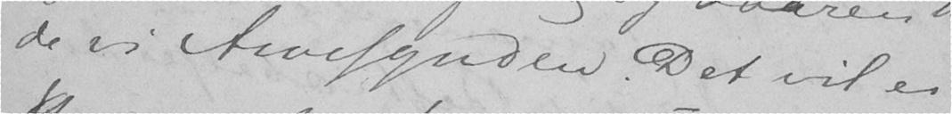de vi Arvesynden. Det vil ei
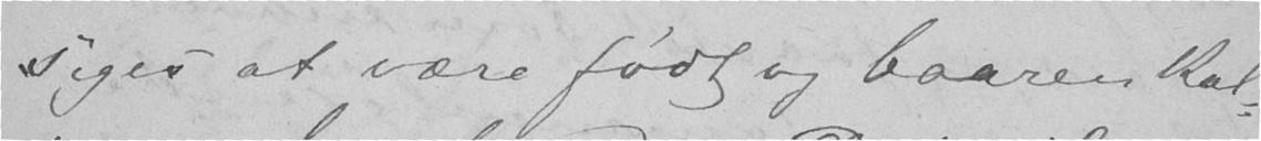siges at være født og baaren kal-
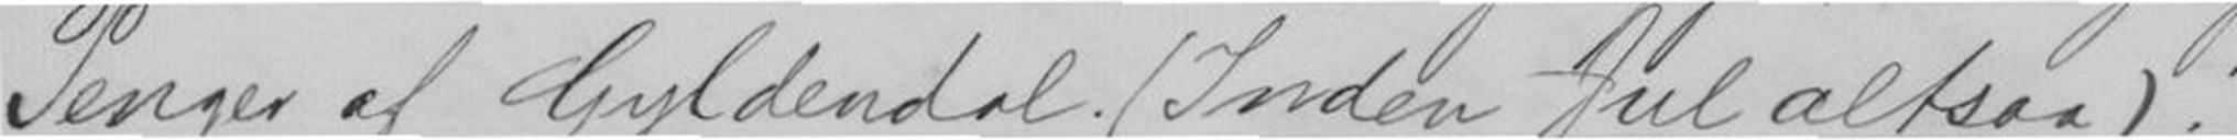Penger af Gyldendal (Inden Jul altsaa).
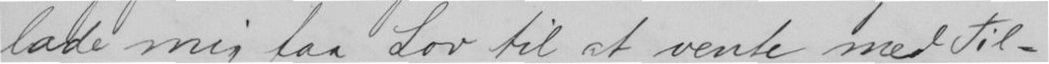lade mig faa Lov til at vente med til-
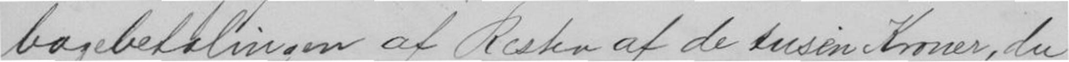bagebetalingen af Resten af de tusen Kroner, du
